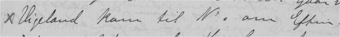x Vigeland kom til N's om Eftm.
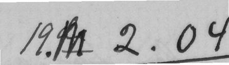19. 2.04
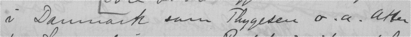i Danmark som Thygesen o. a. Atter
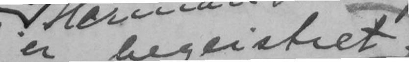er begeistret.
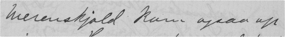Werenskjold kom ogsaa op
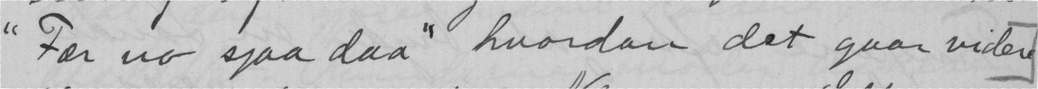"Fær no sjaa daa" hvordan det gaar videre
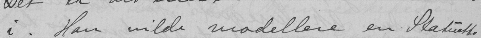i. Han vilde modellere en Statuette
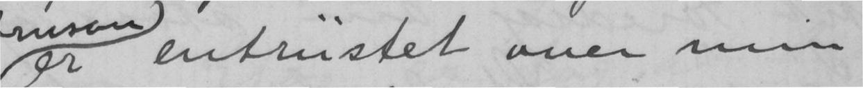er entrüstet over min
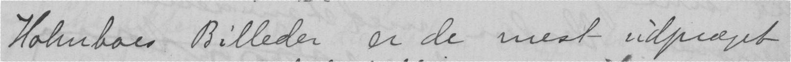Holmboes Billeder er de mest udpræget
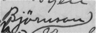Brørnson
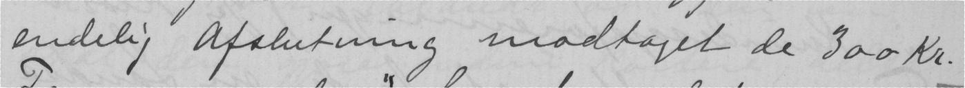endelig Afslutning modtaget de 300 Kr.
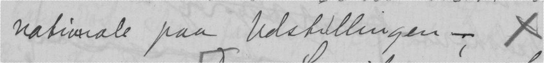nationale paa Udstillingen - x
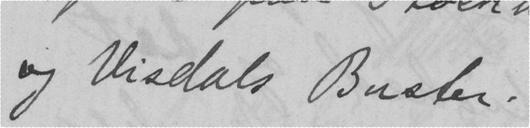og Visdals Buster.
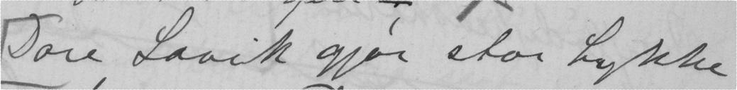Dore Lavik gjør stor Lykke
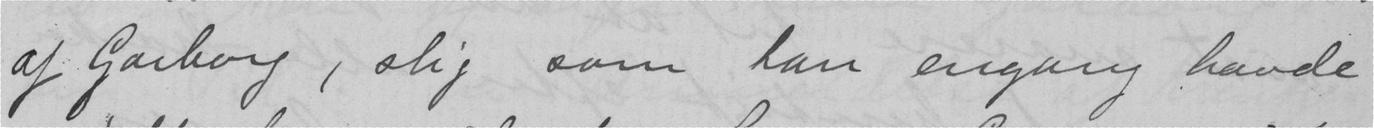af Garborg, slig som han engang havde
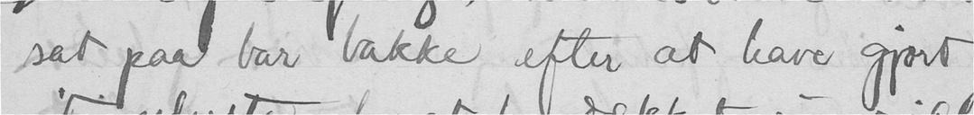sat paa bar vakke efter at have gjort
